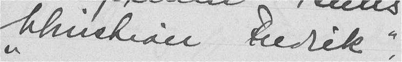"Christian Fredrik",
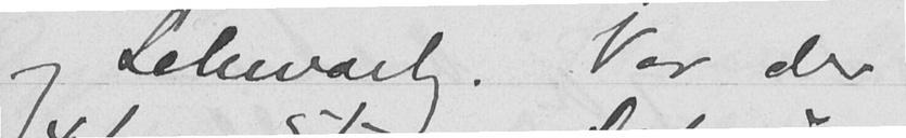og Schwartz. Var der
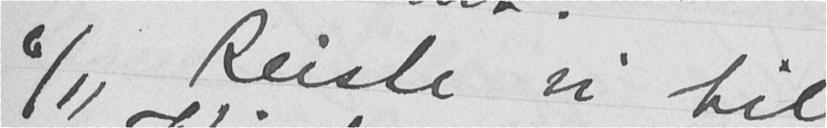6/11 Reiste vi til
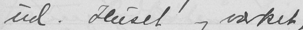ud. Huset og værket,
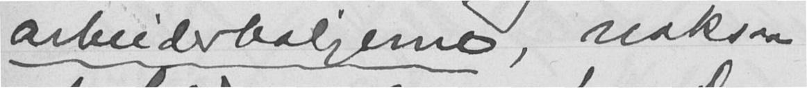arbeiderboligerne, noksaa
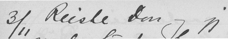3/11 Reiste Don og jeg
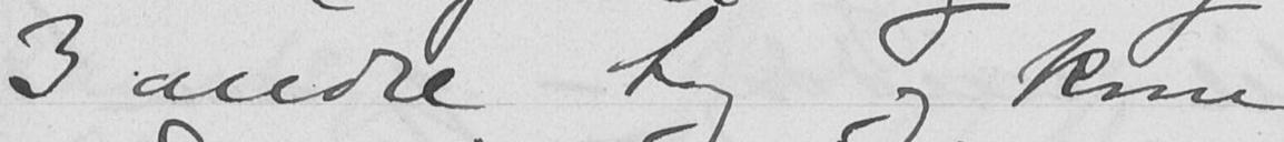3 andre tog og kom
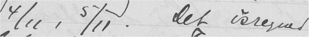4/11, 5/11. Det øsregned
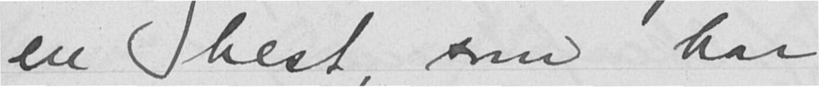en hest, som har
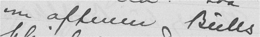om aftenen Bulls
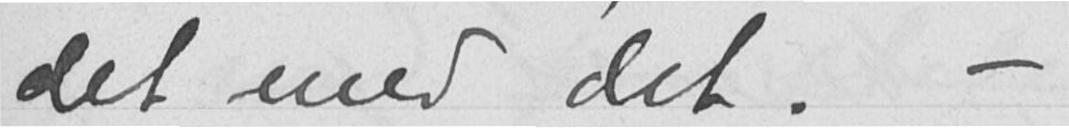det med det. -
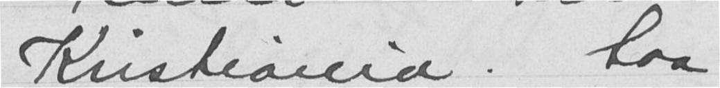Kristiania. Saa
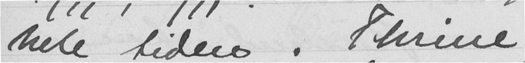hele tiden. Thrine
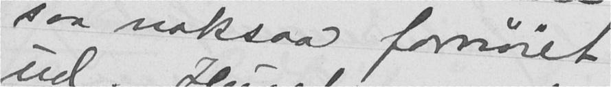saa noksaa fornøiet
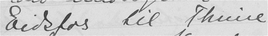Eidsfos til Thrine
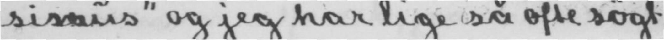simus» og jeg har lige så ofte søgt
